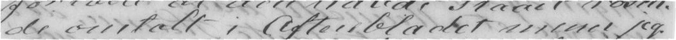de omtalt i Aftenbladet mener jeg.
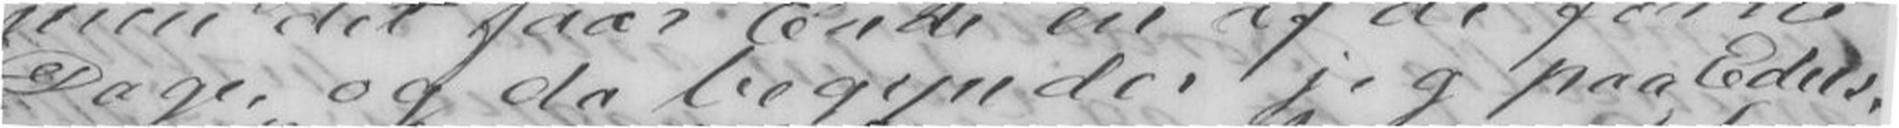Dage, og da begynder jeg paa Eders,
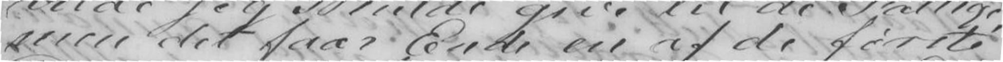men det faar Ende en af de første
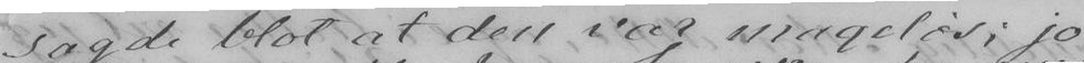sagde blot at den var mageløs; jo
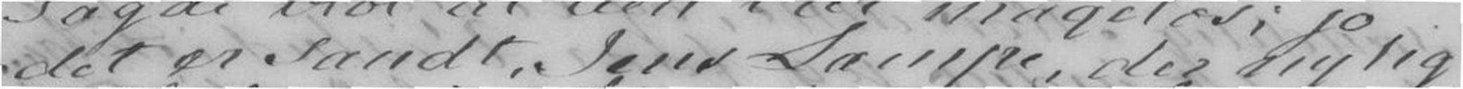det er Sandt, Jens Lampe, der nylig
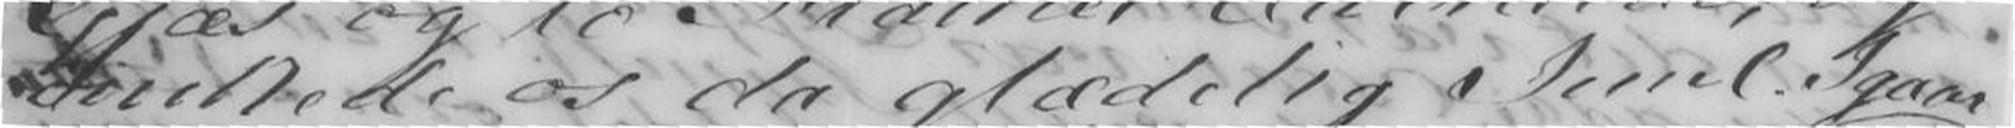ønskede os da glædelig Juul. Igaar
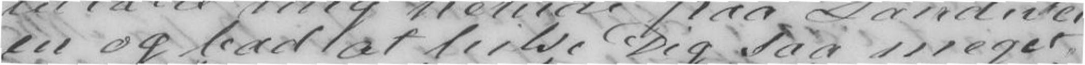en og bad at hilse Dig saa meget,
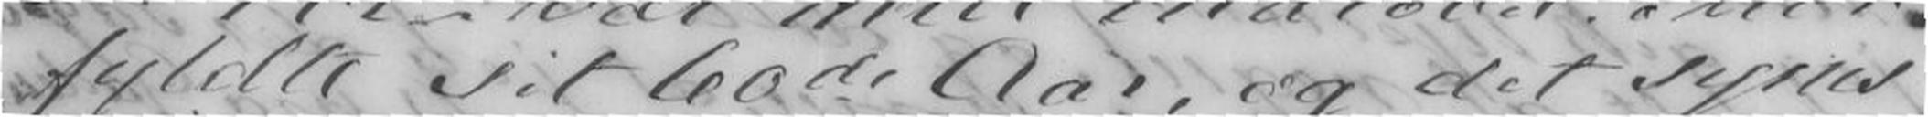fyldte sit 60 Aar, og det synes
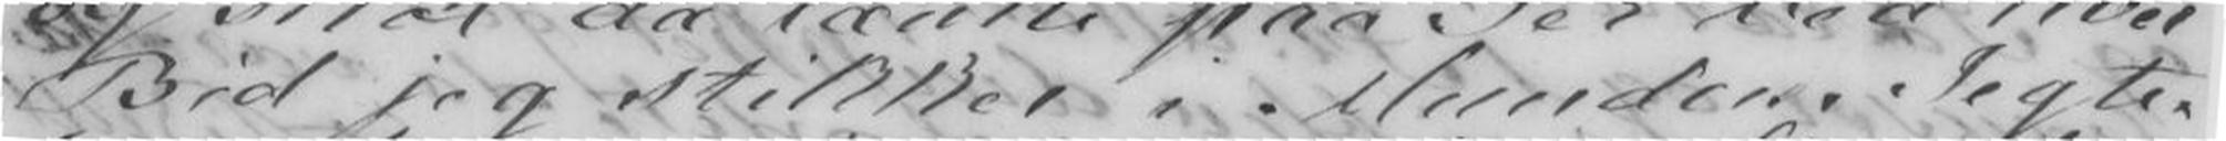Bid jeg stikker i Munden. Jeg te-
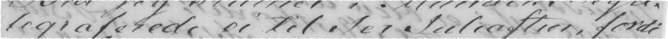legraferede ei til Jer Juleaften, fordi
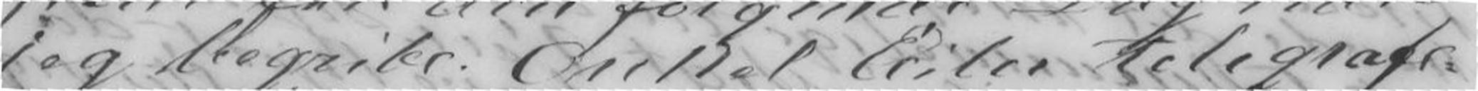jeg begribe Onkel Eiler Telegrafe-
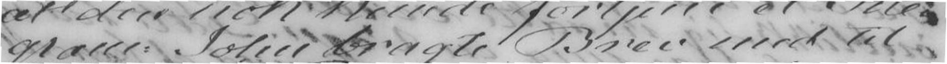gram. John bragte Brev med til
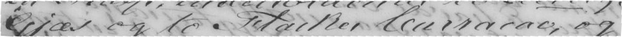Gjæs og to Flasker Curracao, og
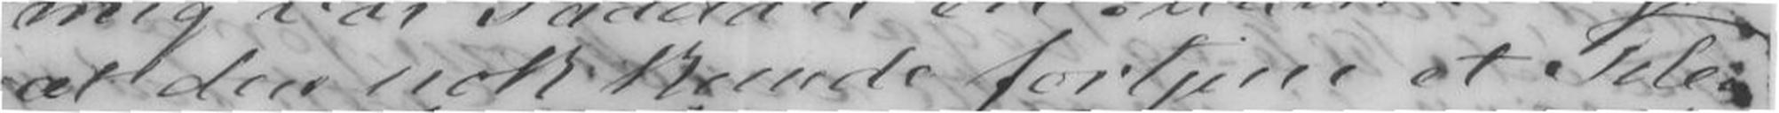at den nok kunde fortjene et Tele-
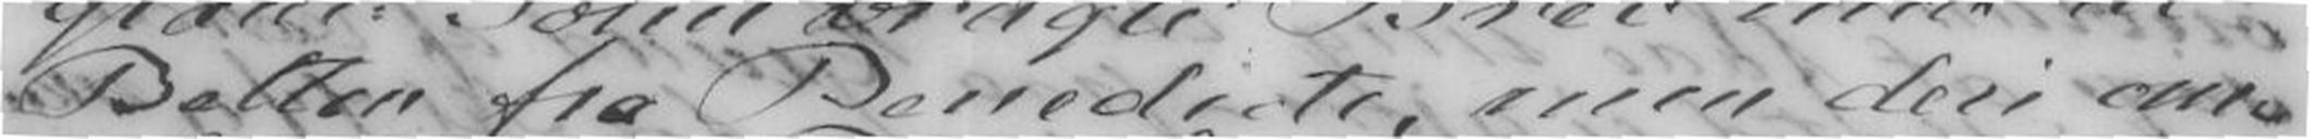Betten fra Benedicte, men deri om-
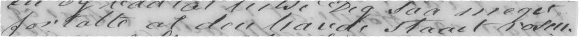fortalte at den havde Staaet rosen-
